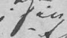i sin
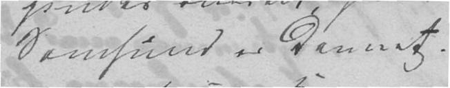Samfund er dannet.
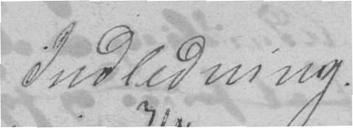Indledning.
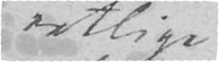retlige
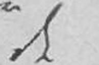de
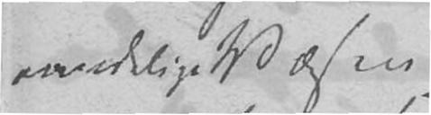aandelige Væsen
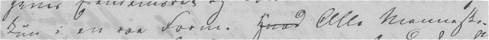kun i en raa Form. Alle Mennesker
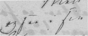ogsaa i sin
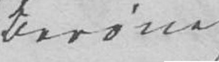berøve
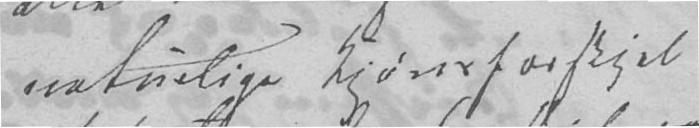naturlige Kjønsforskjel
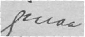maa
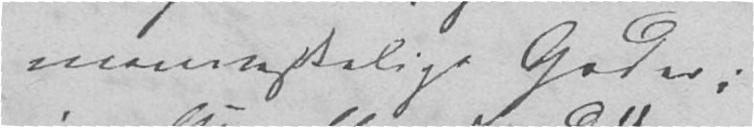menneskelige Goder:
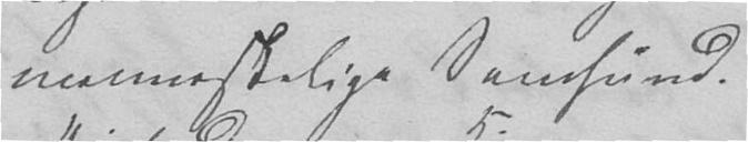menneskelige Samfund.
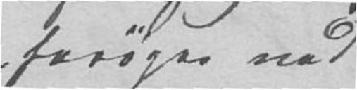forøges med
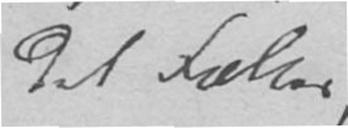det Fælles,
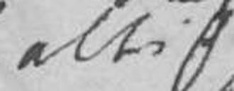altid
